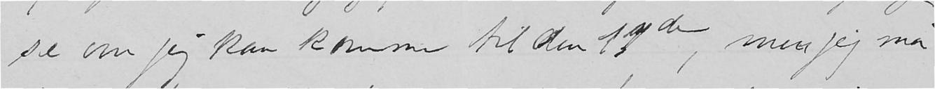se om jeg kan komme til den 17de, men jeg må
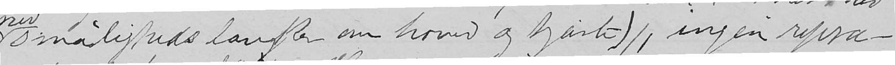småligheds lænker om hoved og hjærte), ingen repræ-
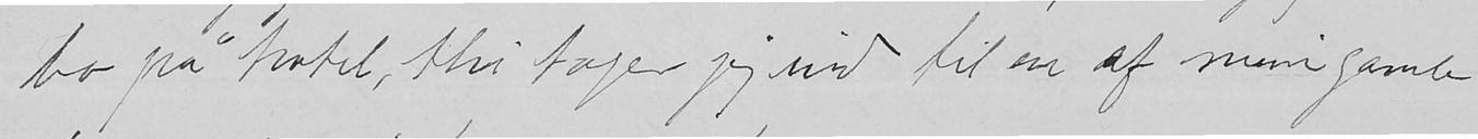bo på hotel, thi tager jeg ind til en af mine gamle
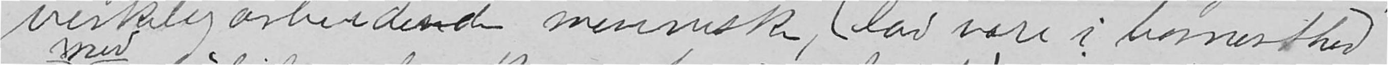virkelighedsarbeidende menneske, (lad være i bornerthed
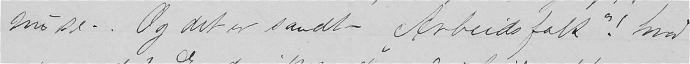nu se. Og det er sandt- "Arbeidsfolk"! hvad
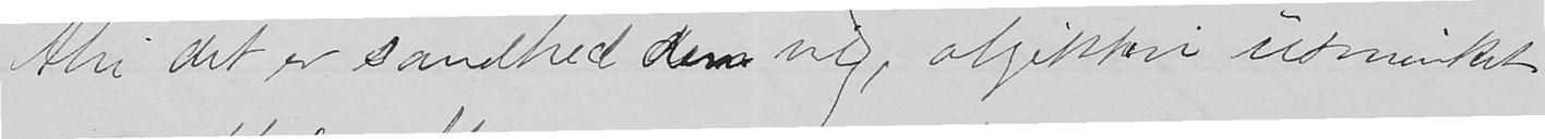thi det er sandhed den vil, objektiv usminket
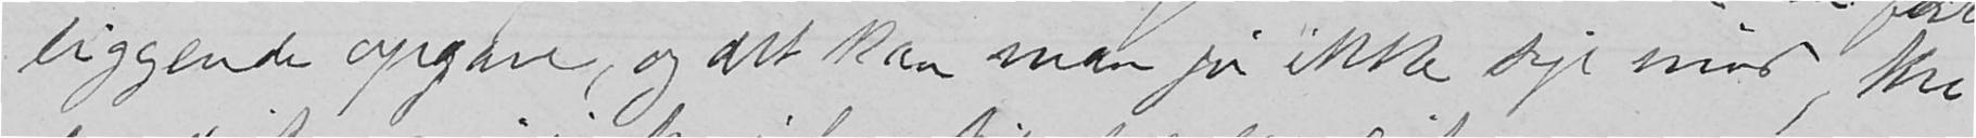liggende opgave, og det kan man jo ikke sige mod, thi
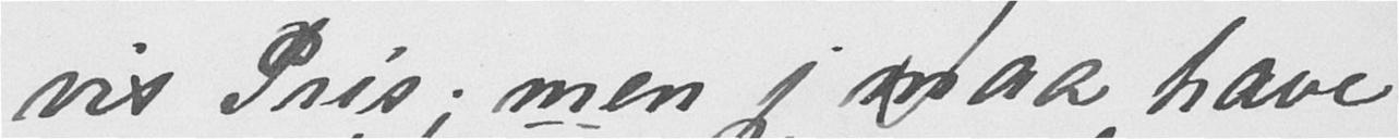vis Pris; men jeg maa have
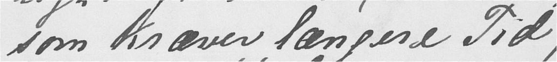som kræver længere Tid,
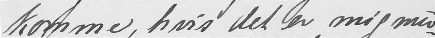komme, hvis det er mig mu-
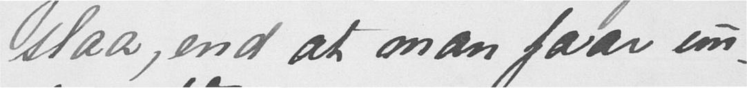slaa, end at man faar un-
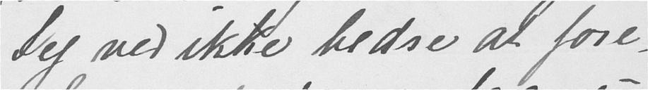Jeg ved ikke bedre at fore-
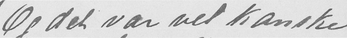Og det var vel kanske
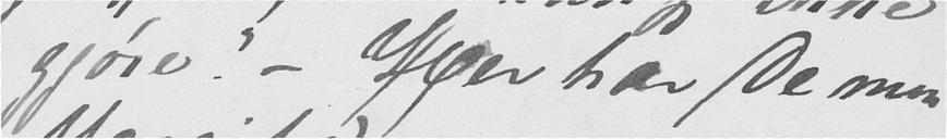gjøre." - Her har De min
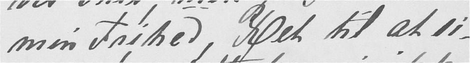min Frihed, Ret til at si-
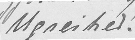Ugreihed.
